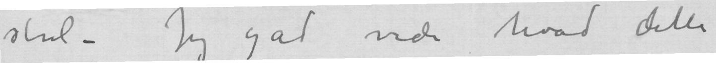stol – Jeg gad vide hvad dette
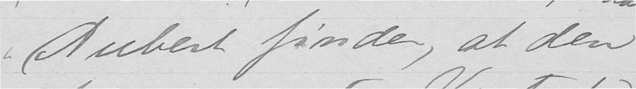Aubert finder, at den
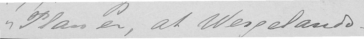Plan er, at Wergelands-
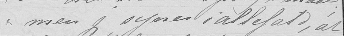men jeg synes iallefald, at
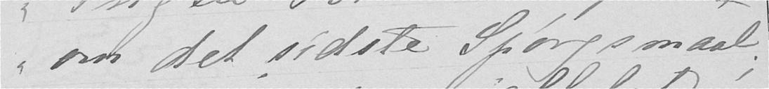om det sidste Spørgsmaal,
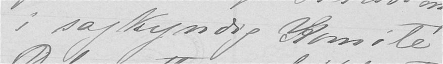i sagkyndige Komité.
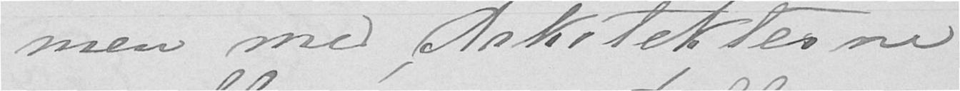men med Arkitekterne
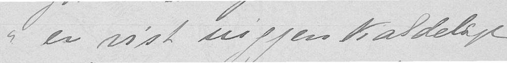er vist uigjenkaldeligt
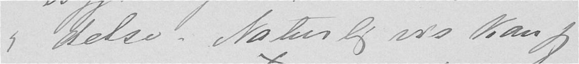delse. Naturligvis kan jeg
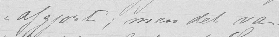afgjort; men det var
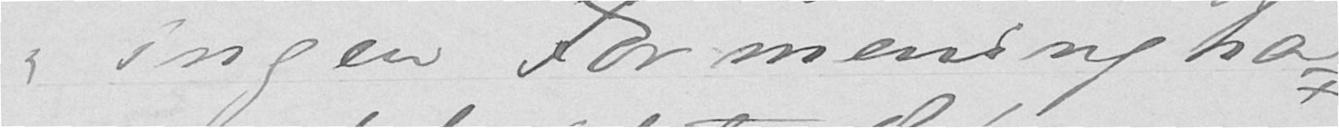ingen Formening ha
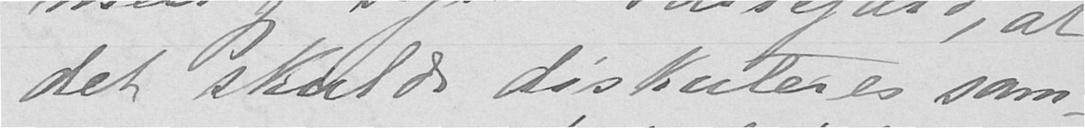det skulde diskuteres sam-
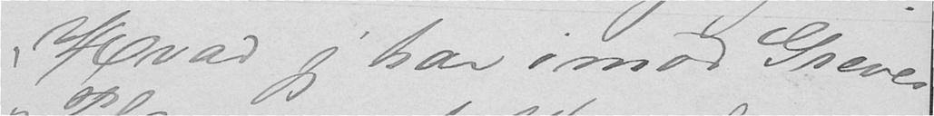Hvad jeg har imod Greves
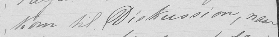kom til Diskussion, naar
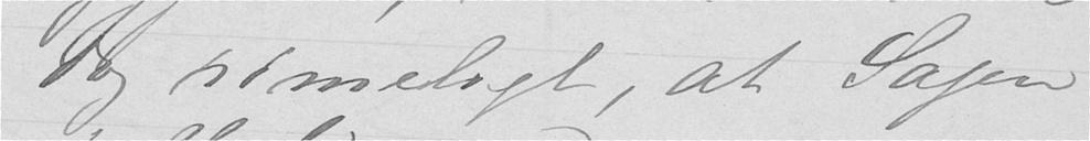dog rimeligt, at Sagen
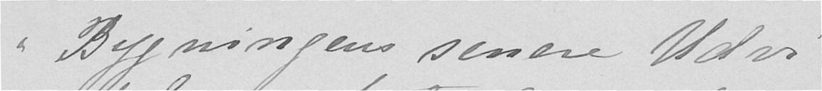Bygningens senere Udvi-
